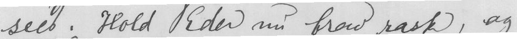sees. Hold Eder nu brav rask, og
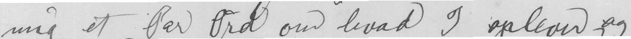mig et Par Ord om hvad I oplever og
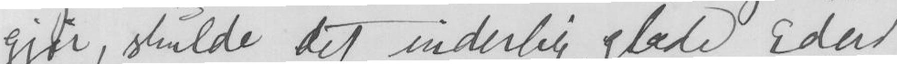gjør, skulde det inderlig glæde Eders
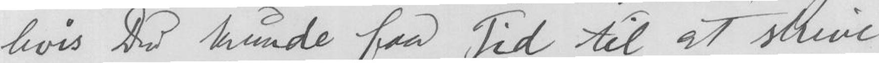hvis Du kunde faa Tid til at skrive
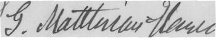G. Matthison=Hansen
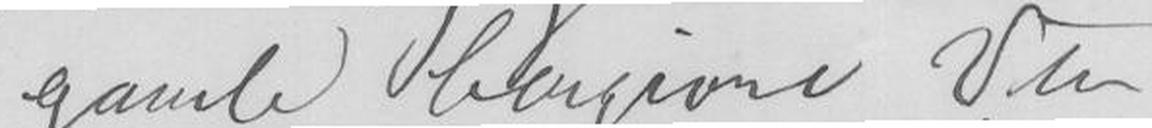gamle hengiven Ven
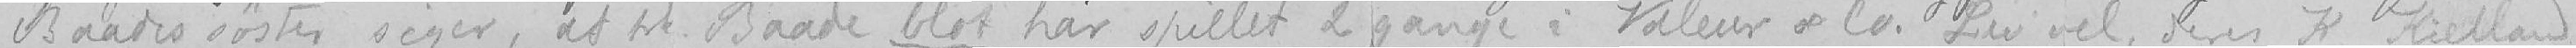Baades søster siger, at frk. Baade blot har spillet 2 gange i Valeur & Co. Lev vel, deres K. Kielland
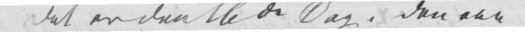Det er den 11de Dag. Den ene
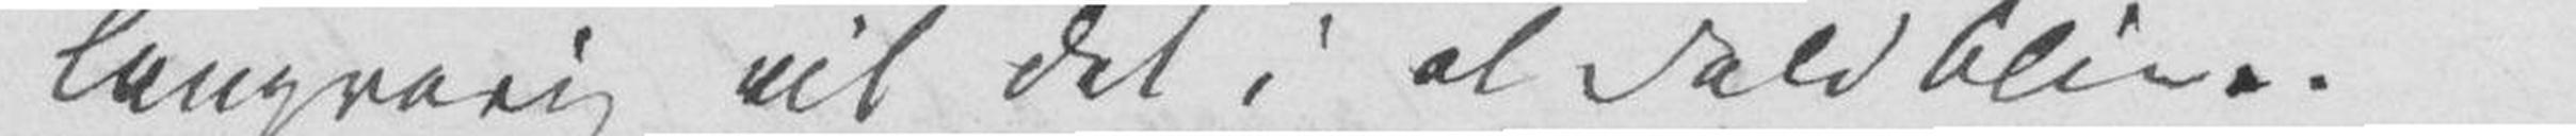langvarig vil det i al Fald blive.
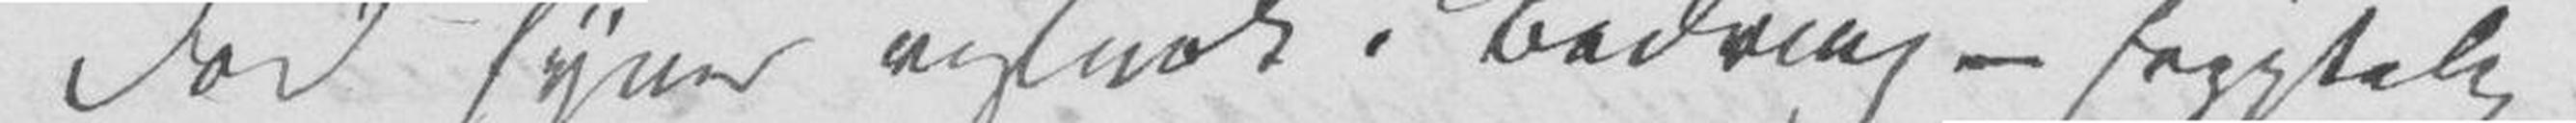Fod synes vistnok i Bedring – frygtelig
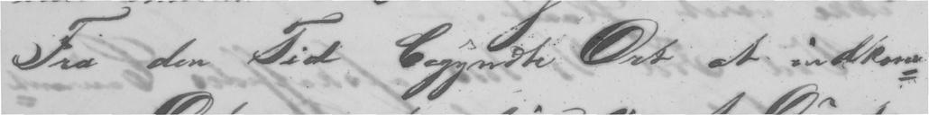Fra den Tid begyndte Ort at indkom-
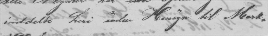inddelte heri uden Hensyn til Mark-
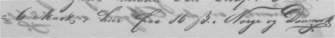= 6 Mark, hver paa 16 ẞ. Norge og Danmark
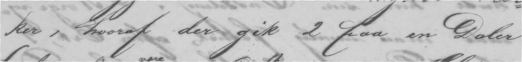ker, hvoraf der gik 2 paa en Daler
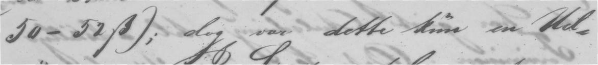50-52 ẞ); dog var dette kun en Ud-
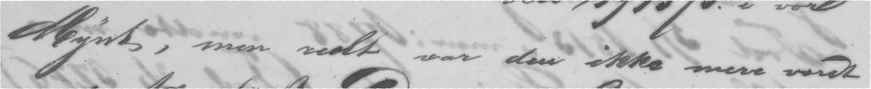Mynt, men reelt var den ikke mere værdt
